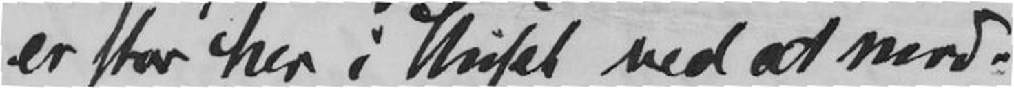er stor her i Huset ved at mod-
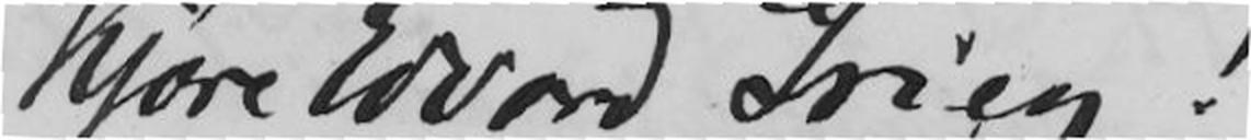Kjære Edvard Grieg!
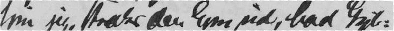som jeg, straks den kom ud, bad Gyl-
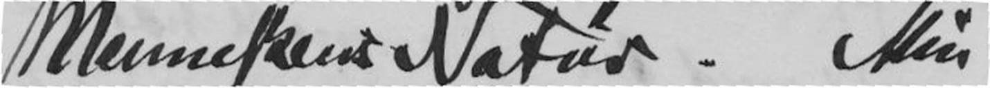Menneskenes Natur. Min
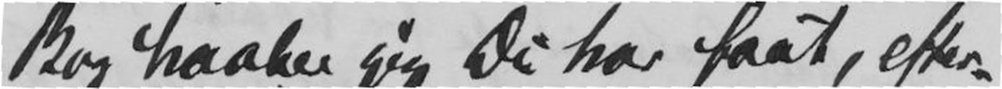Bog haaber jeg Du har faat, efter-
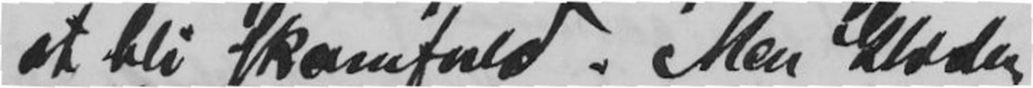at bli skamfuld. Men Glæden
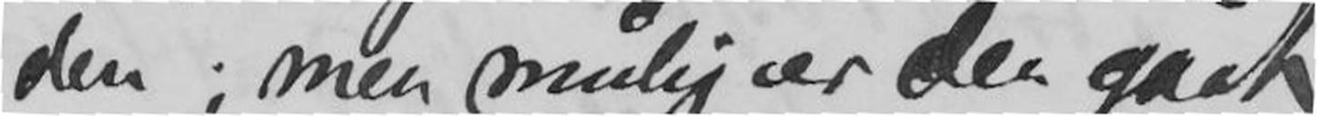den; men mulig er den gaat
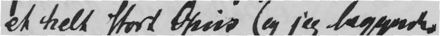et helt stort Opus og jeg begynder
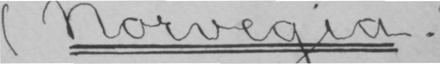(Norvegia.)
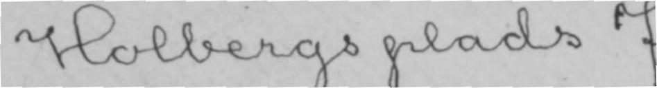Holbergs plads 7.
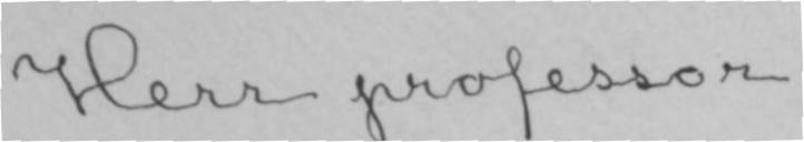Herr professor
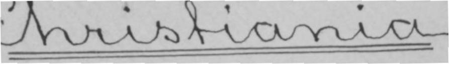Christiania.
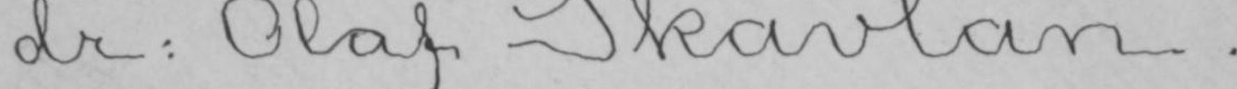dr: Olaf Skavlan.
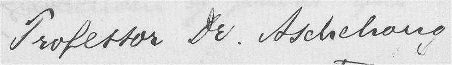Professor Dr. Aschehoug
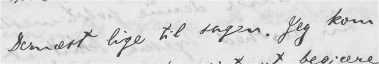Dernæst lige til sagen. Jeg kom
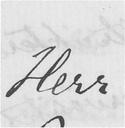Herr
